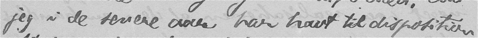jeg i de senere aar har havt til disposition.
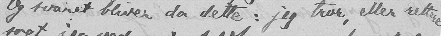Og svaret bliver da dette: jeg tror, eller rettere
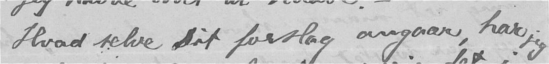Hvad selve Dit forslag angaar, har jeg
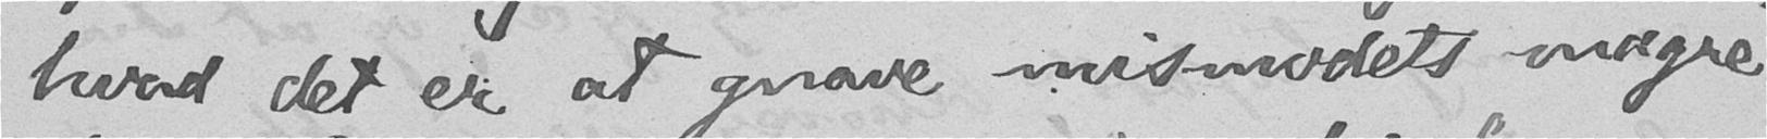hvad det er at grave mismodets magre
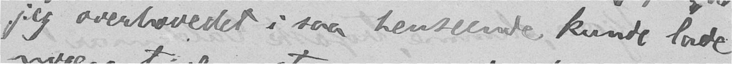jeg overhovedet i saa henseende kunde lade
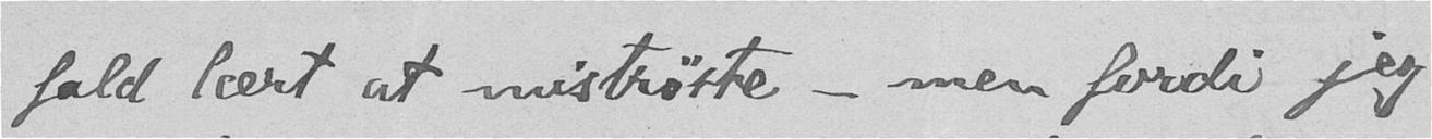fald lært at mistrøste – men fordi jeg
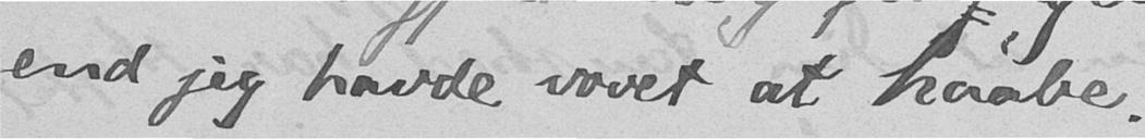end jeg havde vovet at haabe. -
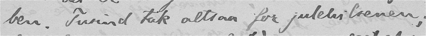ben. Tusind tak altsaa for julehilsenen;
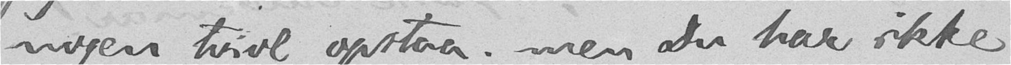nogen tvivl opstaa; men Du har ikke
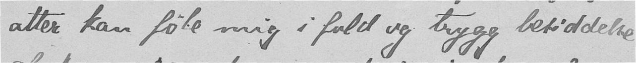atter kan føle mig i fald og trygg besiddelse
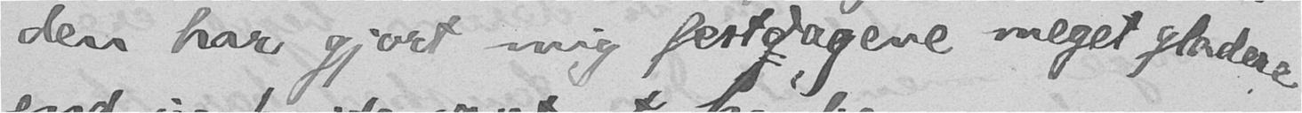den har gjort mig festdagene meget gladere
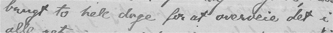brugt to hele dage for at overveie det i
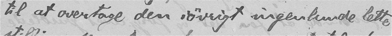til at overtage den iøvrigt ingelunde lette
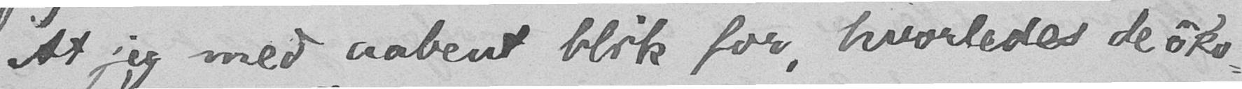At jeg med aabent blik for, hvorledes de øko-
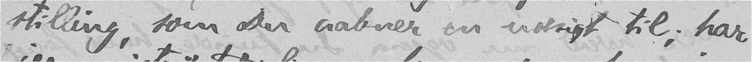stilling, som Du aabner en udsigt til; har
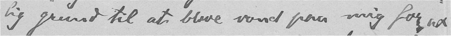lig grund til at blive vond paa mig for at
